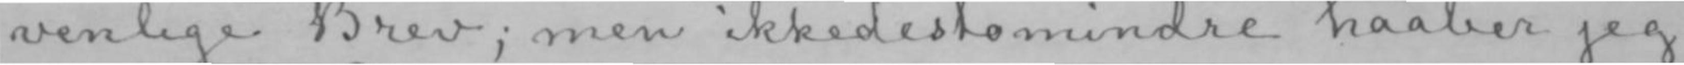venlige Brev; men ikkedestomindre haaber jeg
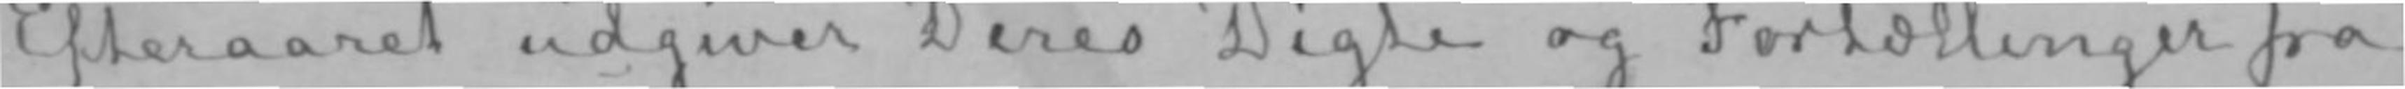Efteraaret udgiver Deres Digte og Fortællinger fra
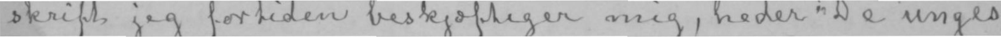skrift jeg fortiden beskjæftiger mig, heder «De unges
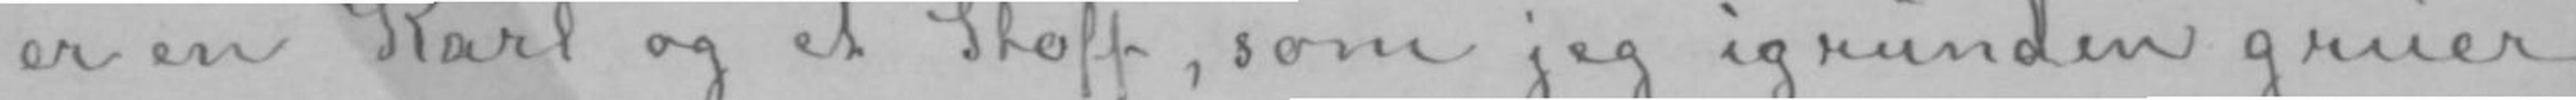er en Karl og et Stoff, som jeg igrunden gruer
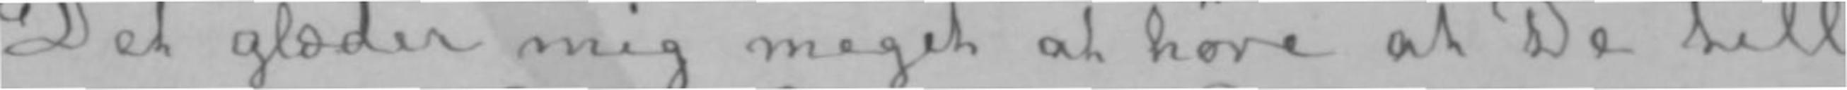Det glæder mig meget at høre at De till
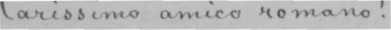Carissimo amico romano!
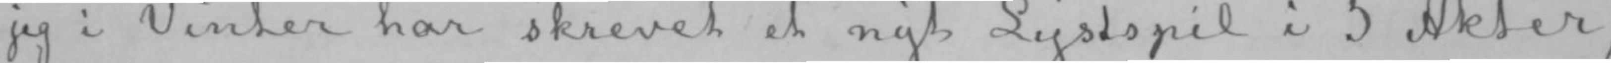jeg i Vinter har skrevet et nyt Lystspil i 5 Akter,
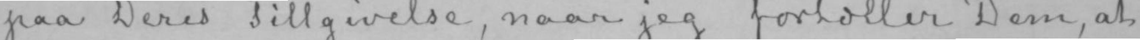paa Deres Tillgivelse, naar jeg fortæller Dem, at
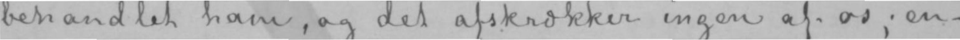behandlet ham, og det afskrækker ingen af os; en-
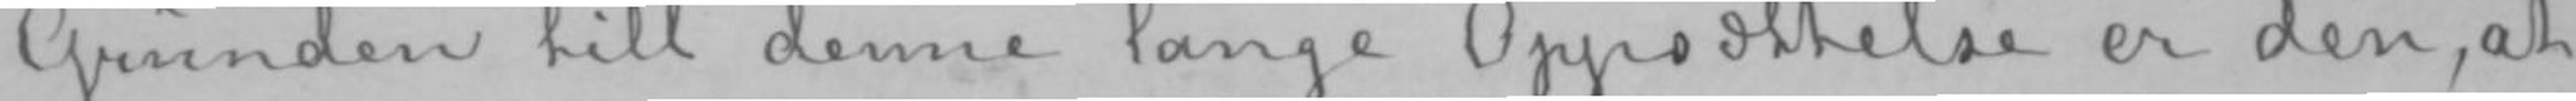Grunden till denne lange Oppsættelse er den, at
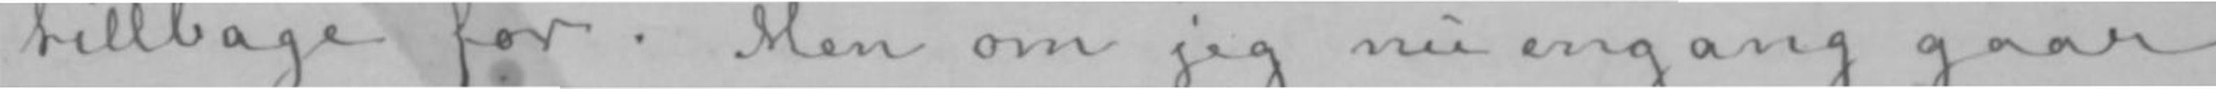tillbage for. Men om jeg nu engang gaar
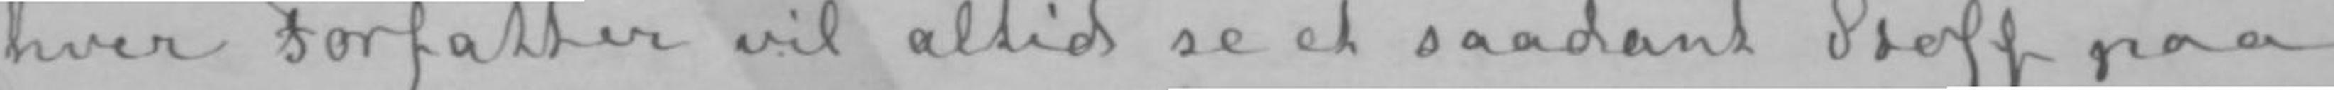hver Forfatter vil altid se et saadant Stoff paa
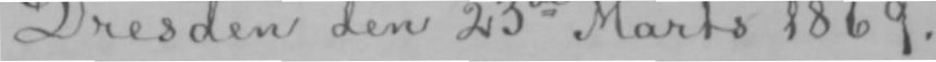Dresden den 23de Marts 1869.
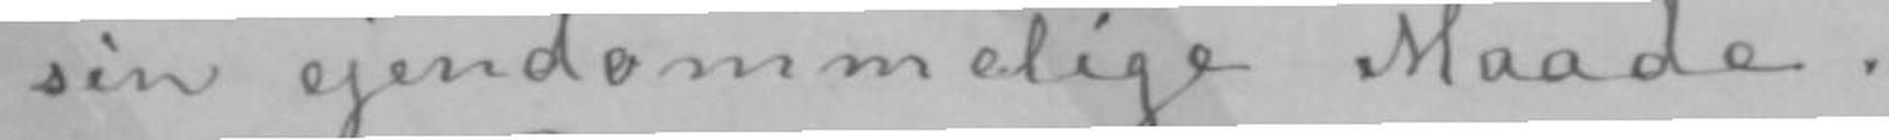sin ejendommelige Maade.
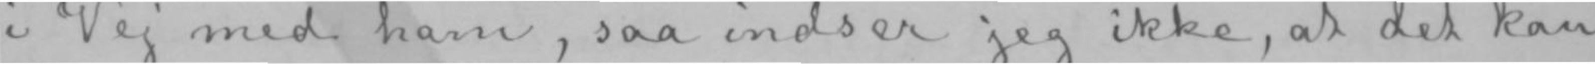i Vej med ham, saa indser jeg ikke, at det kan
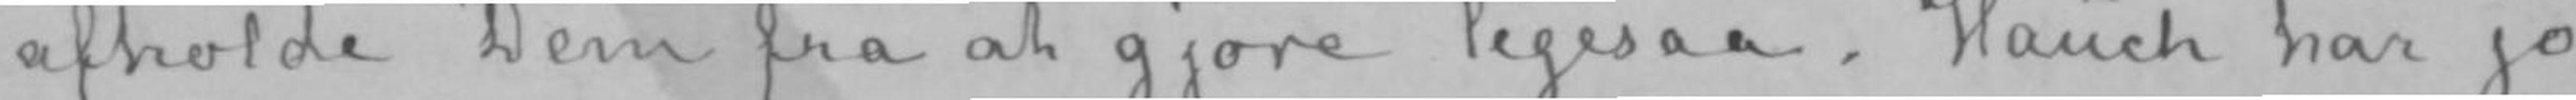afholde Dem fra at gjøre ligesaa. Hauch har jo
